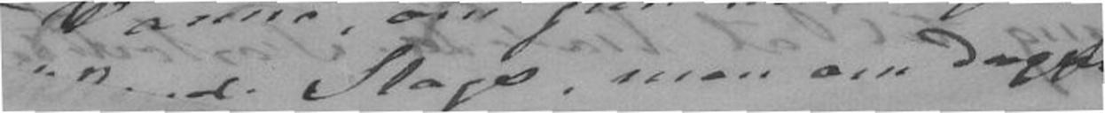Slags, men om Dagen
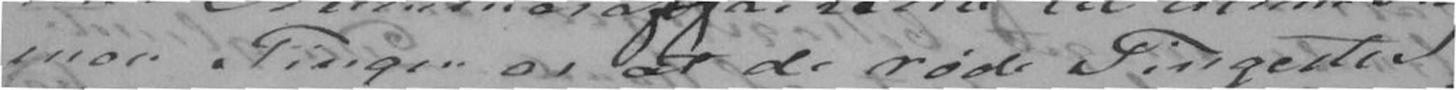men Tingen er at de røde Tingester
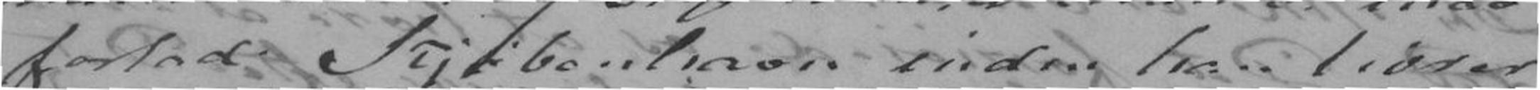forlade Kjøbenhavn inden han hører
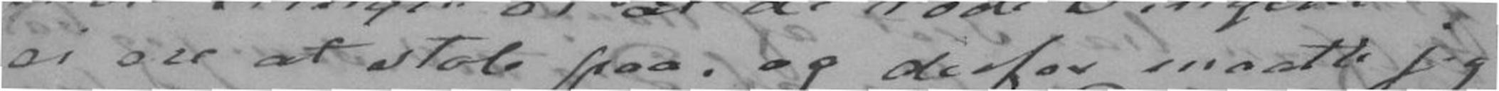ei ere at stole paa, og derfor maatte jeg
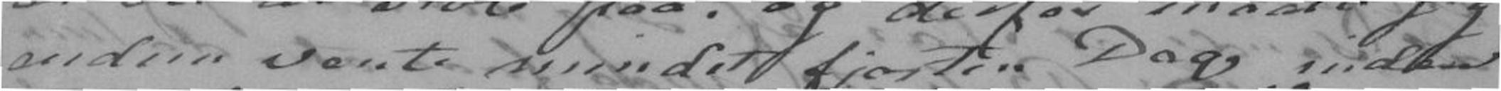endnu vente mindst fjorten Dage inden
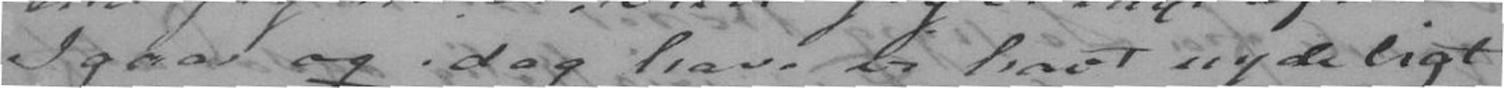Igaar og idag have vi havt nydeligt
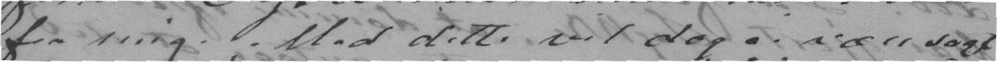fra mig. Med dette vil dog ei være sagt
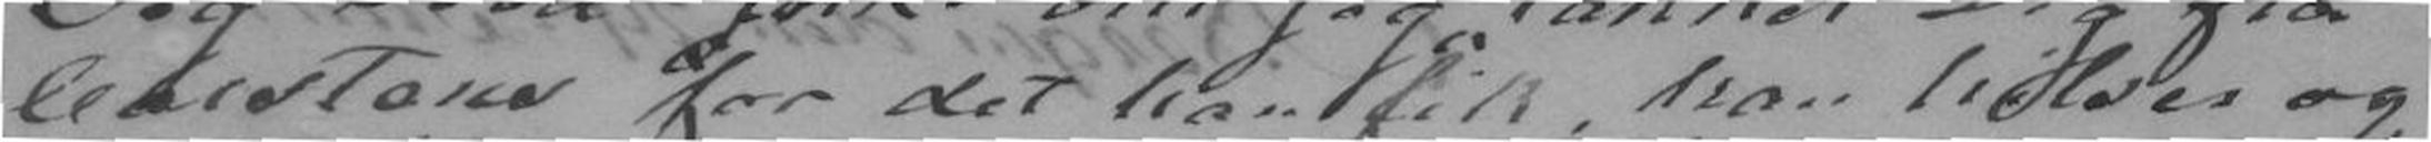Carstens for det han fik, han hilser og
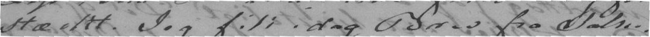stærkt. Jeg fik idag Brev fra John,
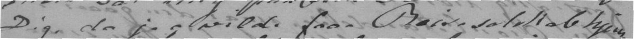Dig da jeg vilde faae Reiseselskab hjem-
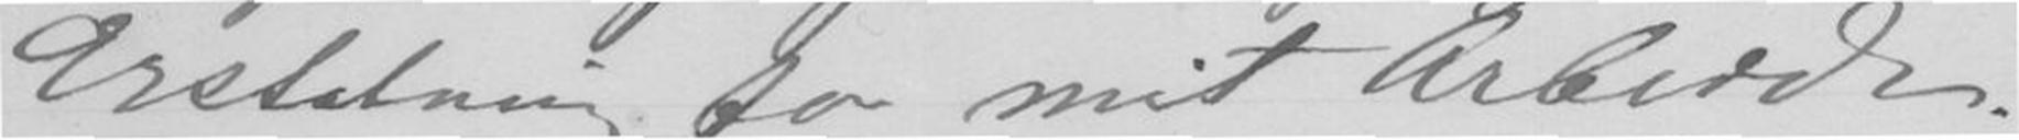Erstatning for mit Arbeide.
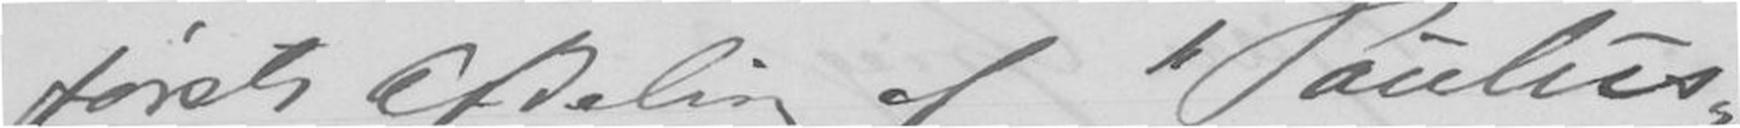første Afdeling af "Paulus"
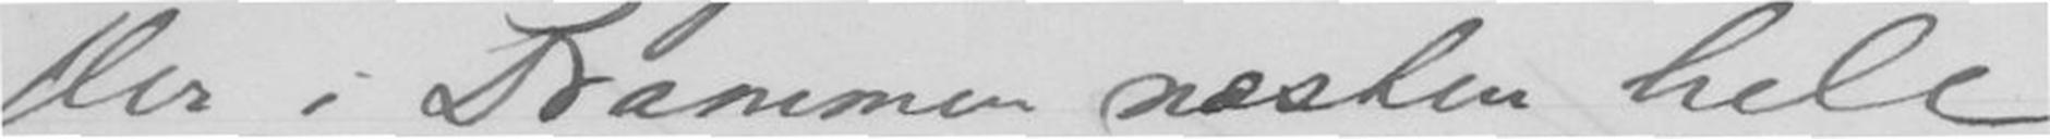Her i Drammen næsten hele
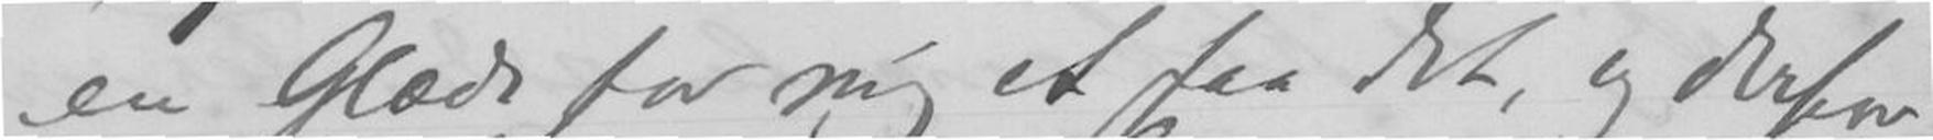en Glæde for mig at faa Dit, og derfor
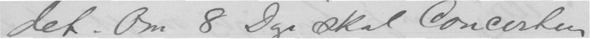Om 8 Dage skal Concerten
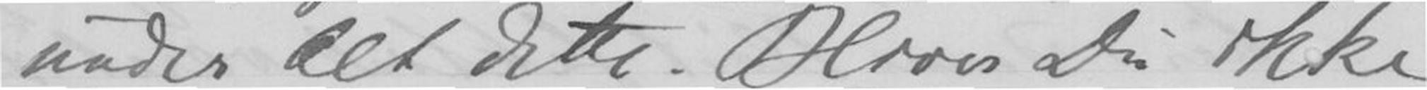under Alt dette. Bliver Du ikke
